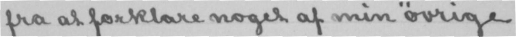fra at forklare noget af min øvrige
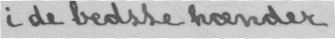i de bedste hænder.
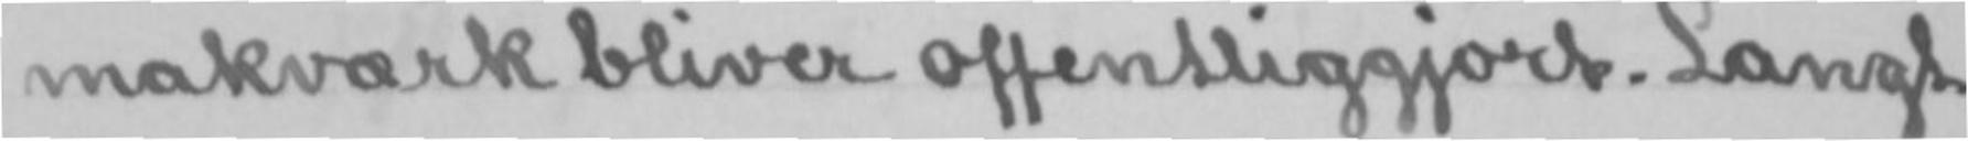makværk bliver offentliggjort. Langt
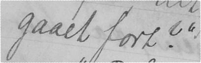gaaet fort?"
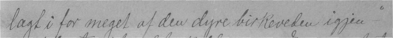lagt i for meget af den dyre birkeveden igjen - "
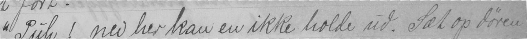"Puh! ned her kan en ikke holde ud. Sæt op døren
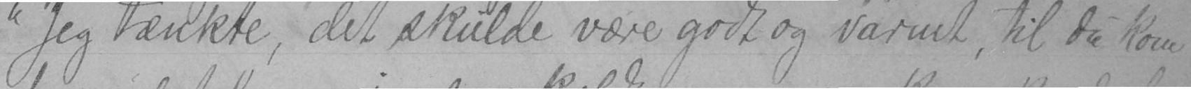"Jeg tænkte, det skulde være godt og varmt, til du kom
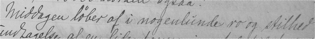Middagen løber af i nogenlunde ro og stilhed
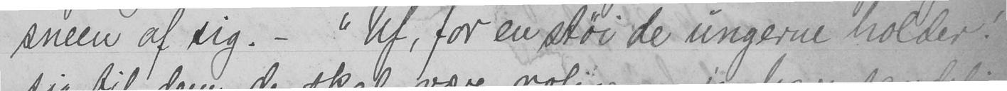sneen af sig. -  "Uf, for en støi de ungerne holder!
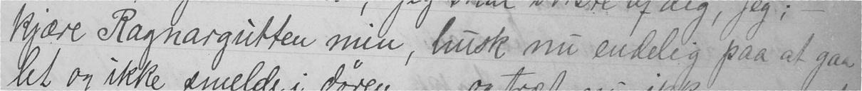kjære Ragnargutten min, husk nu endelig paa at gaa
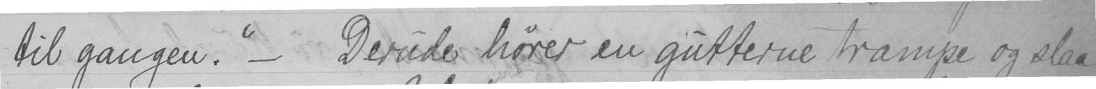til gangen." - Dernede hører en gutterne trampe og slaa
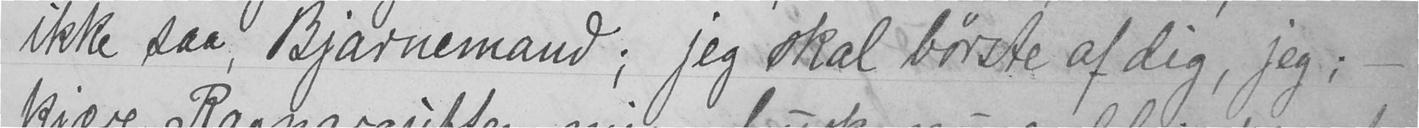ikke saa, Bjarnemand; jeg skal børste af dig, jeg; -
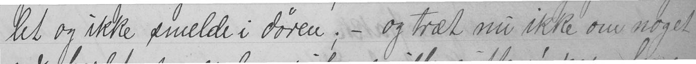let og ikke smelde i døren; - og træt nu ikke om noget
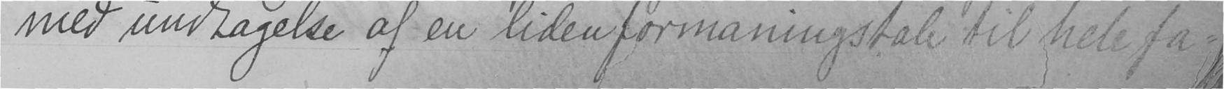med undtagelse af en liden formaningstale til hele fa-
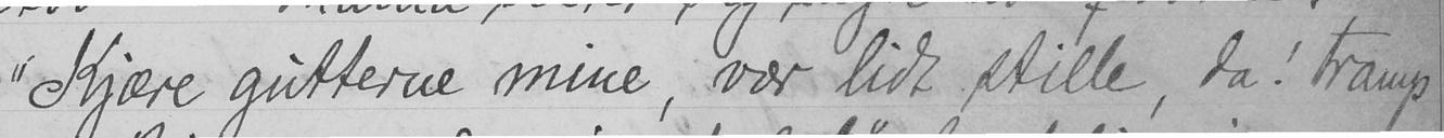"Kjære gutterne mine, vær lidt stille, da! tramp
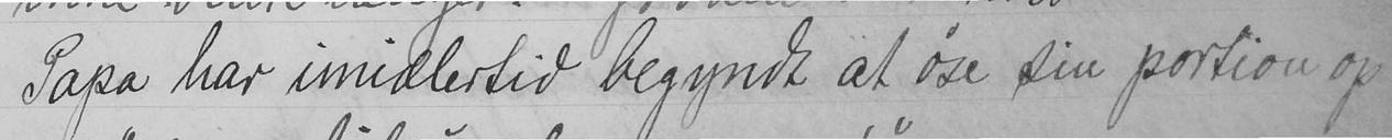Papa har imidlertid begyndt at øse sin portion op
